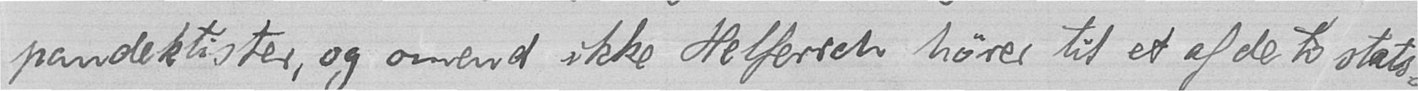pandektister, og omend ikke Helferich hører til et af de to stats-
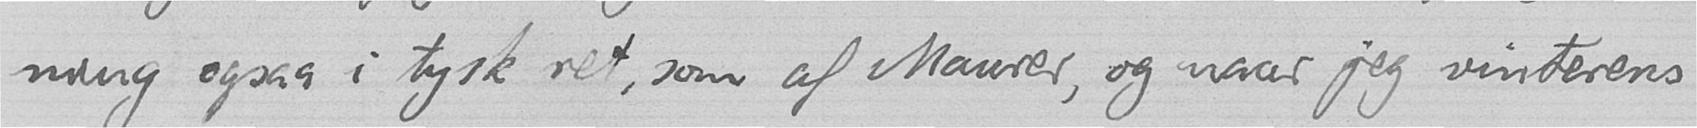ning ogsaa i tysk ret, som af Maurer, og naar jeg vinterens
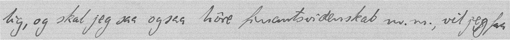lig, og skal jeg saa ogsaa høre finantsvidenskab m. m., vil jeg faa
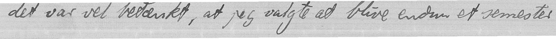det var vel betænkt, at jeg valgte at blive endnu et semester
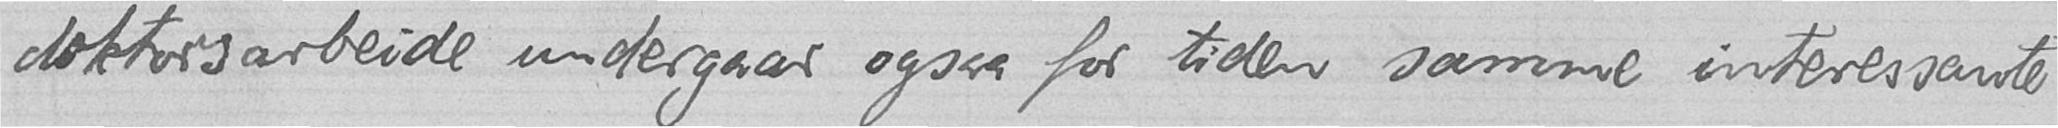doktorsarbeide undergaar ogsaa for tiden samme interessante
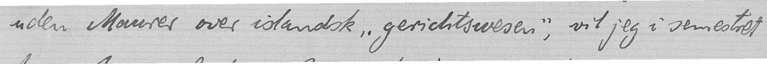uden Maurer over islandsk "gerichtswesen", vil jeg i semestret
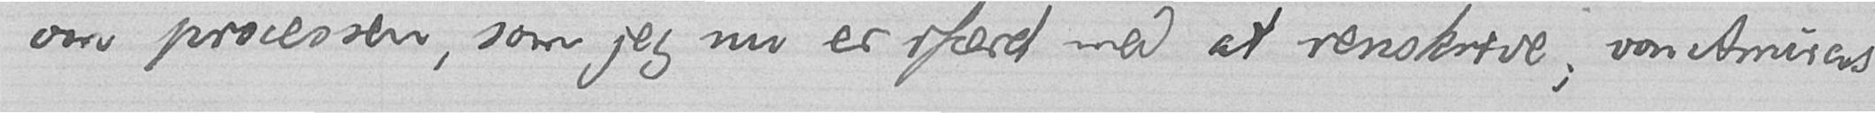om processen, som jeg nu er ifærd med at renskrive; von Amiras
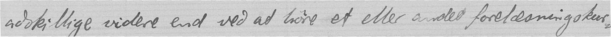adskillige videre end ved at høre et eller andet forelæsningskur-
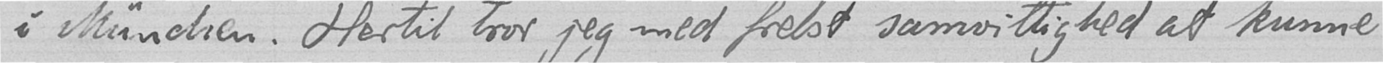i München. Hertil tror jeg med frelst samvittighed at kunne
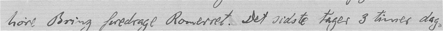høre Brinz foredrage Romerret. Det sidste tager 3 timer dag-
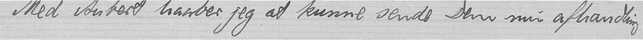Med Aubert haaber jeg at kunne sende Dem min afhandling
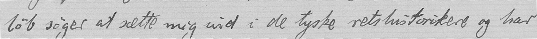løb søger at sætte mig ind i de tyske retshistorikere og har
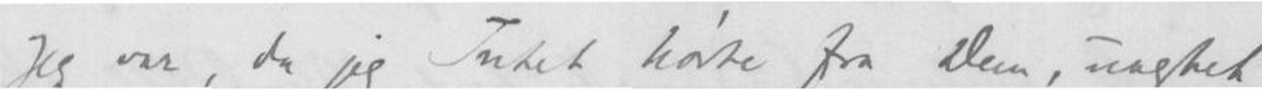Jeg var, da jeg intet hørte fra Dem, vagtet
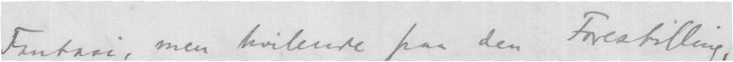Fantasi, men hvilende paa den Forestilling,
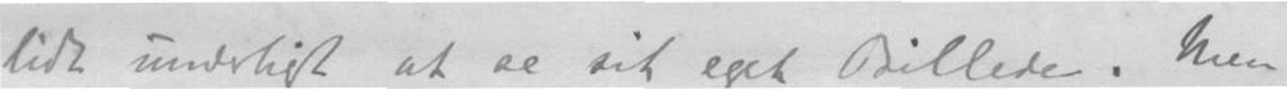lidt underligt at se sit eget Billede. Men
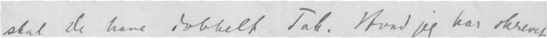skal De have dobbel Tak. Hvad jeg har skrevet
